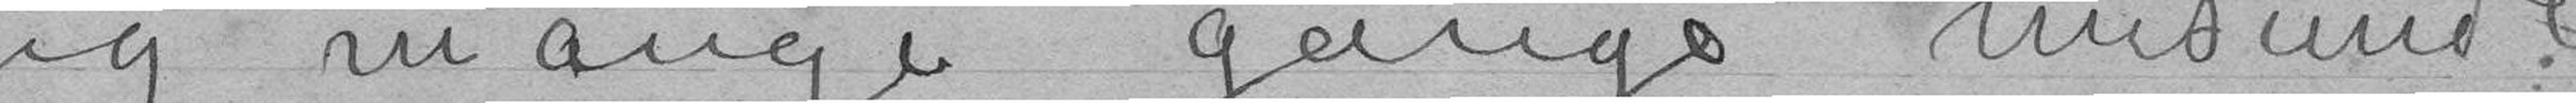jeg mange gange misunde
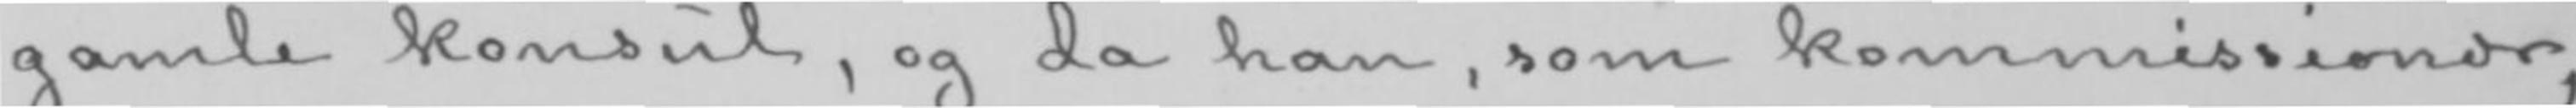gamle konsul, og da han, som kommissionær,
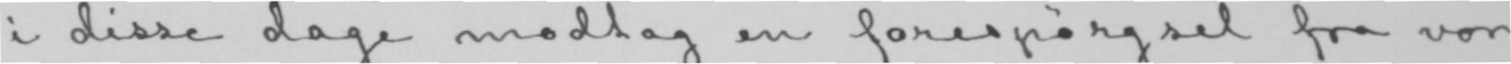i disse dage modtog en forespørgsel fra vor
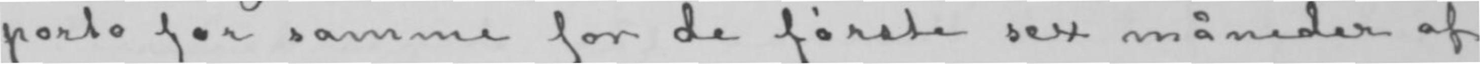porto for samme for de første sex måneder af
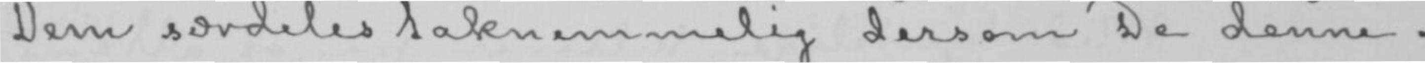Dem særdeles taknemmelig dersom De denne-
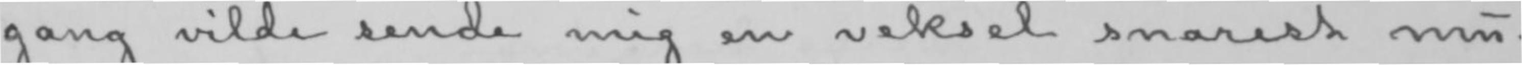gang vilde sende mig en veksel snarest mu-
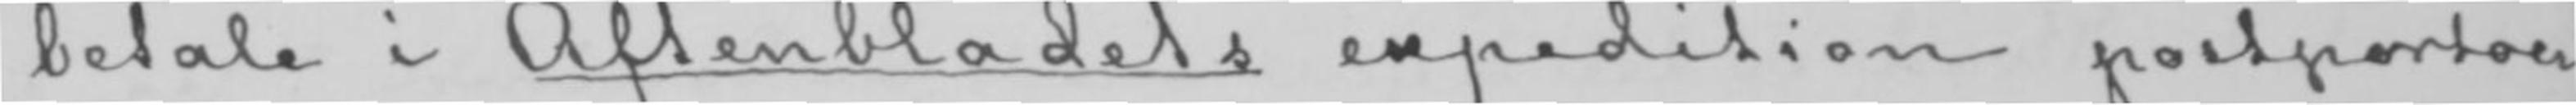betale i Aftenbladets expedition postportoen
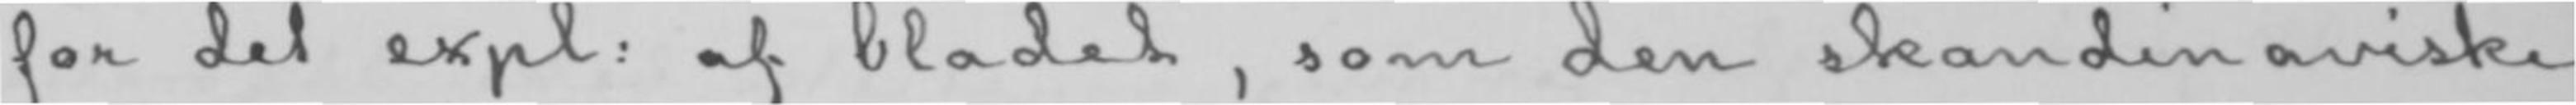for det expl: af bladet, som den skandinaviske
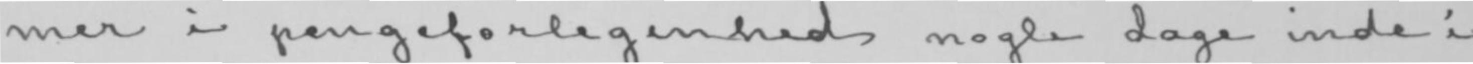mer i pengeforlegenhed nogle dage inde i
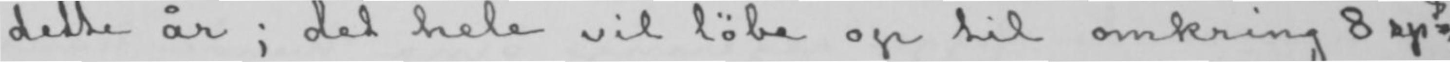dette år; det hele vil løbe op til omkring 8 spd.
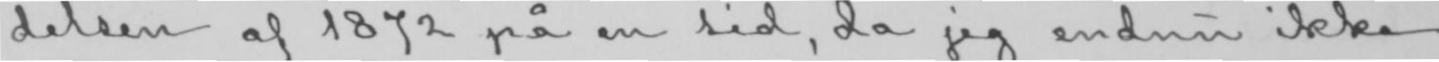delsen af 1872 på en tid, da jeg endnu ikke
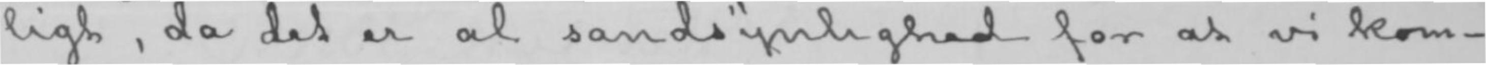ligt, da det er al sandsynlighed for at vi kom-
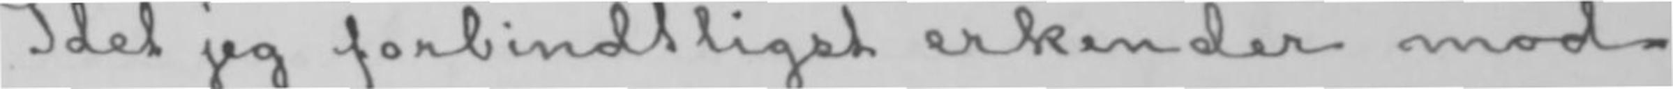Idet jeg forbindtligst erkender mod-
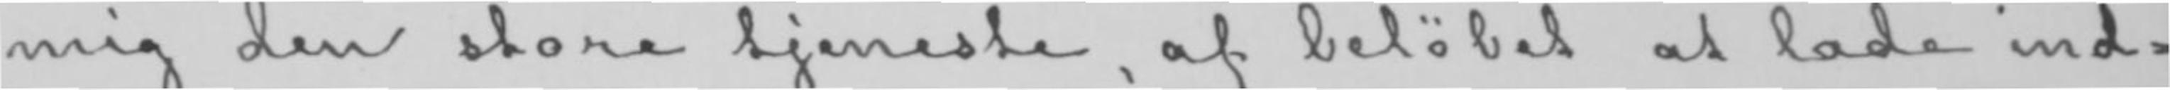mig den store tjeneste, af beløbet at lade ind-
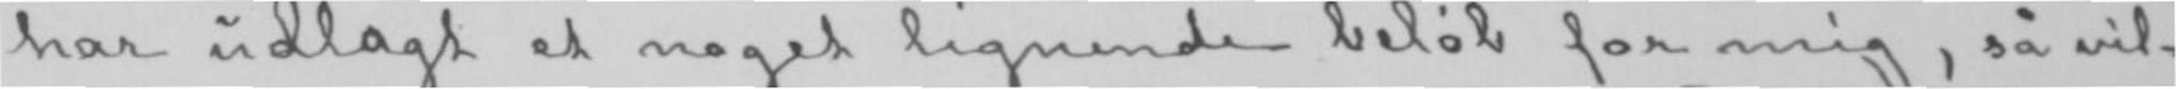har udlagt et noget lignende beløb for mig, så vil-
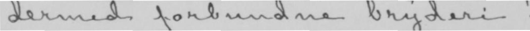dermed forbundne bryderi!
